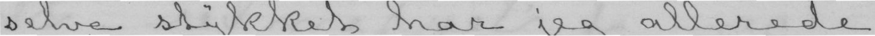selve stykket har jeg allerede
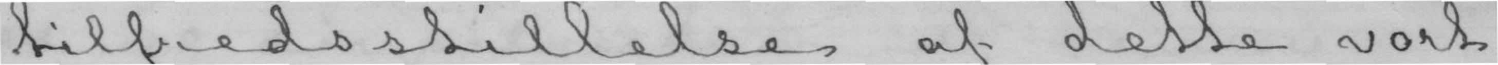tilfredsstillelse af dette vort
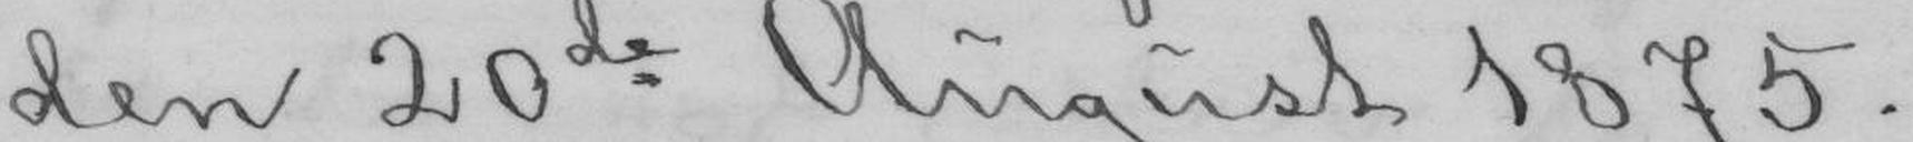den 20de August 1875.
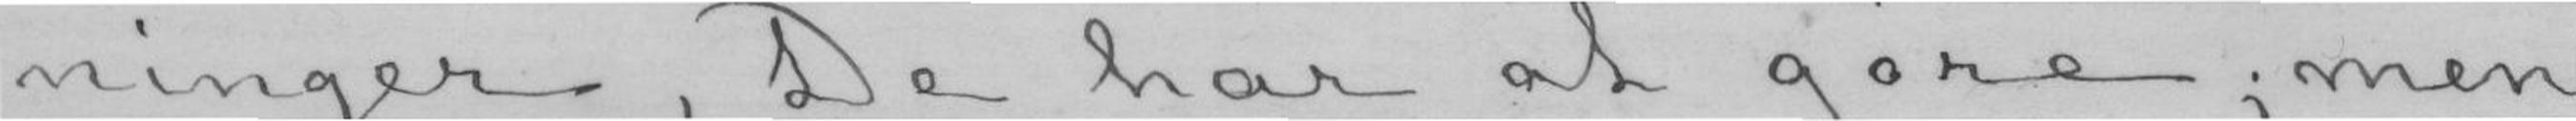ninger, De har at gøre; men
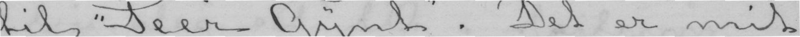til "Peer Gynt". Det er mit
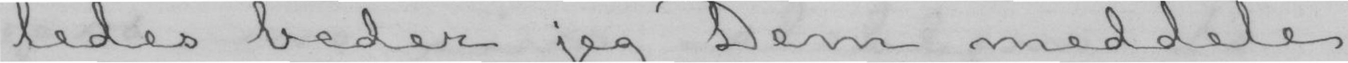ledes beder jeg Dem meddele
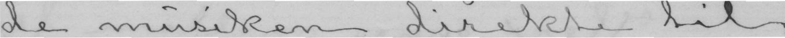de musiken direkte til
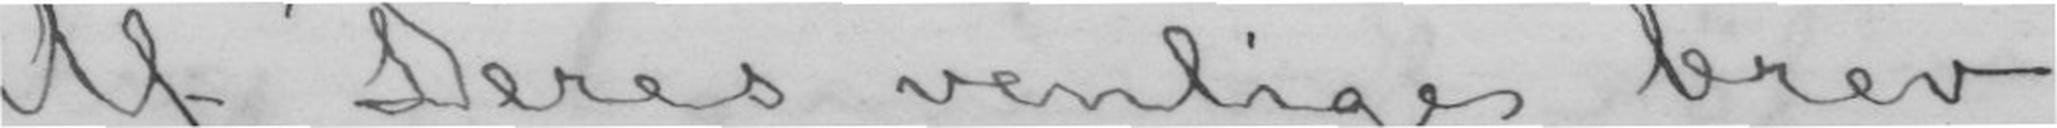Af Deres Venlige brev
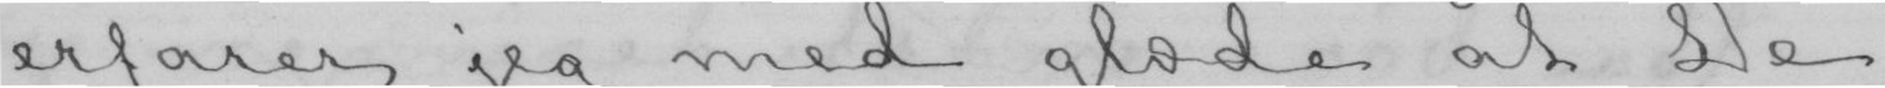erfarer jeg med glæde at De
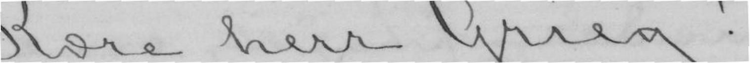Kære herr Grieg!
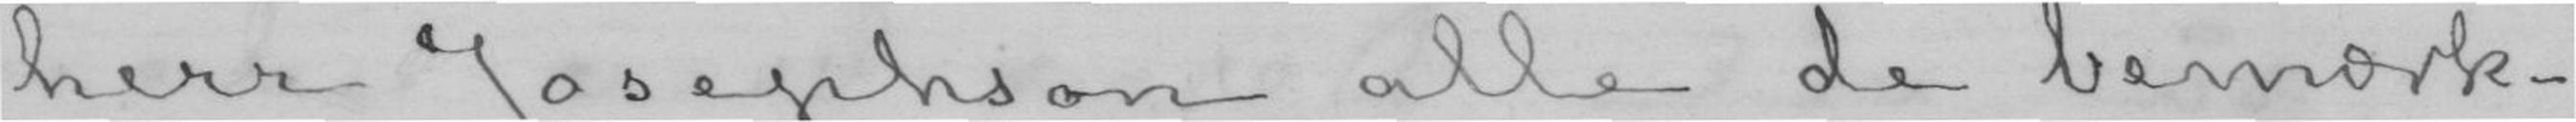herr Josephson alle de bemærk-
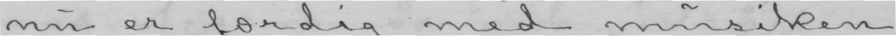nu er færdig med musiken
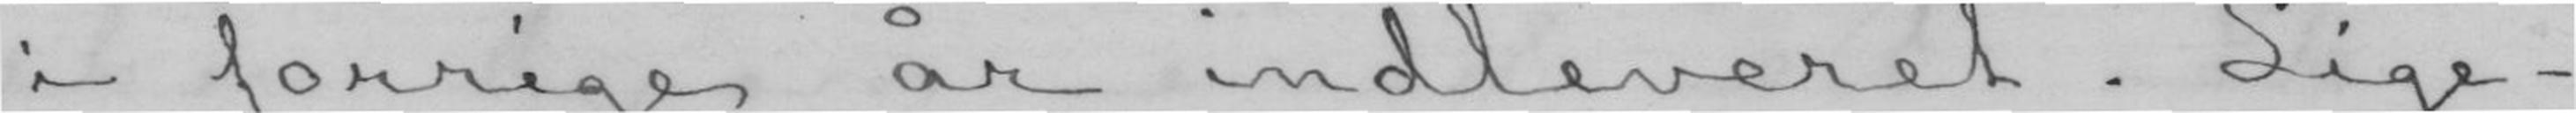i forrige år indleveret. Lige-
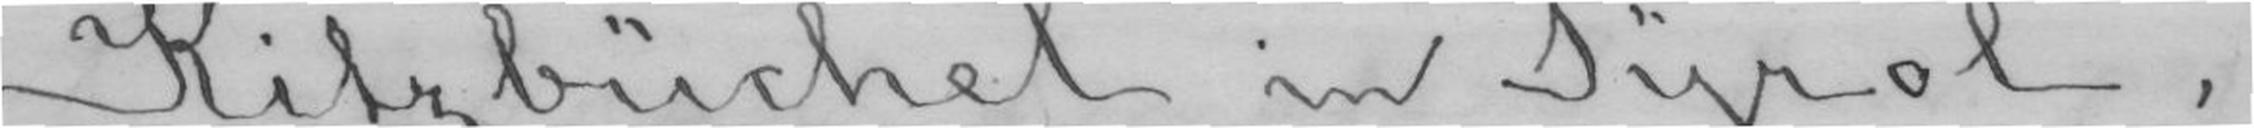Kitzbüchel in Tyrol,
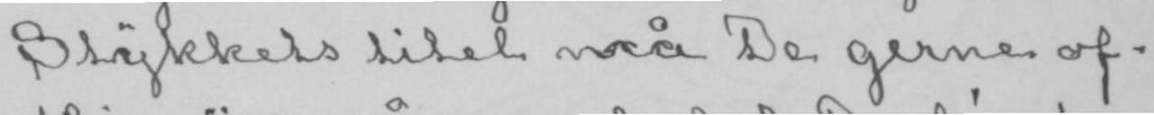Stykkets titel må De gerne of-
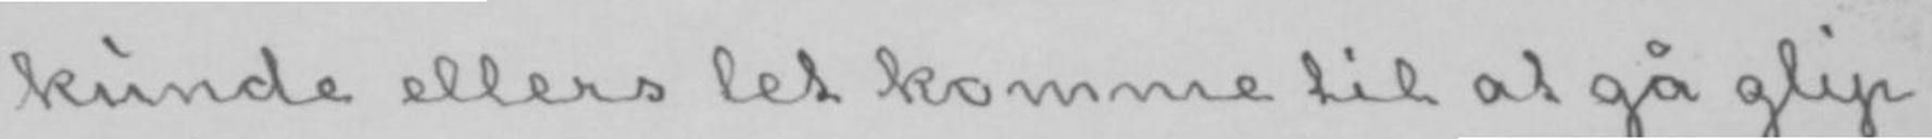kunde ellers let komme til at gå glip
